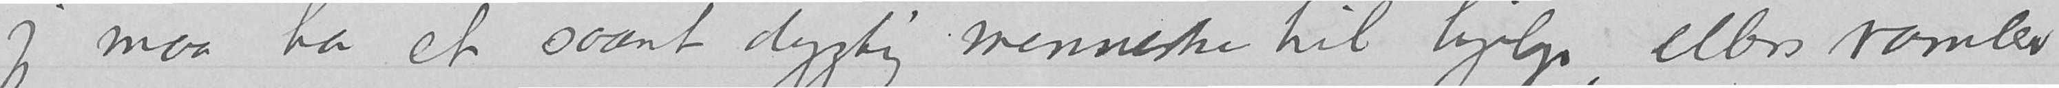jeg maa ha et svært dygtig menneske til hjelp, ellers ramler
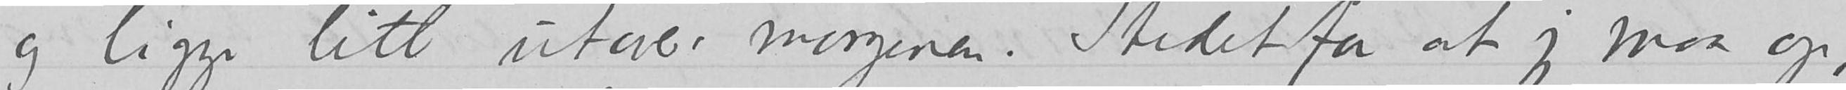og ligge litt utover morgenen. I stedet for at jeg maa op,
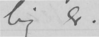lig er.
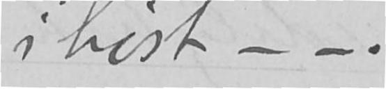gjøre i høst - -.
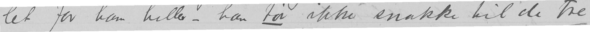let for ham heller – han tør ikke snakke til de tre
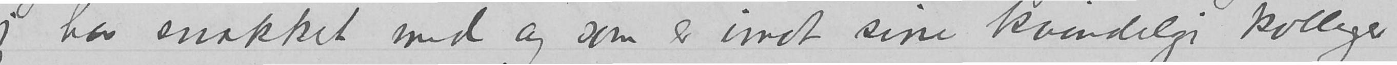jeg har snakket med og som er imot sine kvindelige kolleger
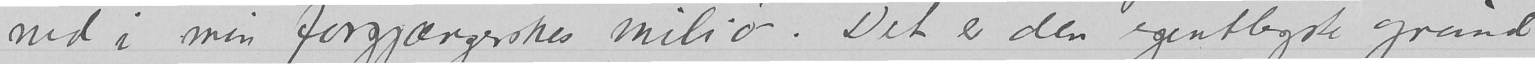ned i min forgjængerskes miliø. Det er den egentligste grund
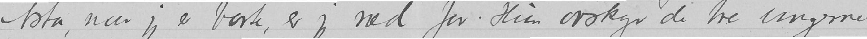Asta, naar jeg er borte, er jeg ræd for. Hun avskyr de tre ungerne
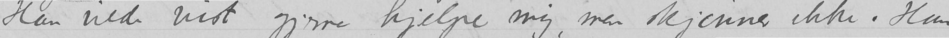Han vilde vist gjerne hjelpe mig, men skjønner ikke. Han
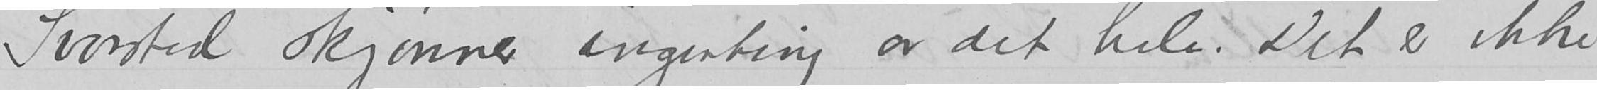Svarstad skjønner ingenting av det hele. Det er ikke
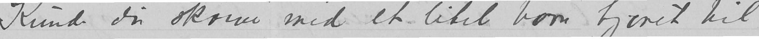Kunde du skrive med et litet barn tjoret til
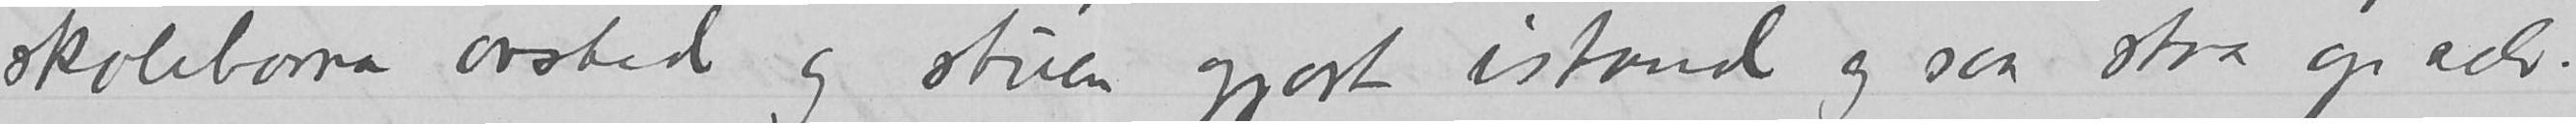skolebarna avsted og stuen gjort istand og saa staa op selv.
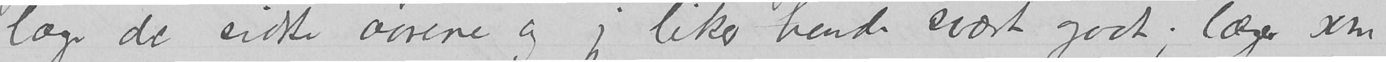læge de sidste aarene og jeg liker hende svært godt; læger som
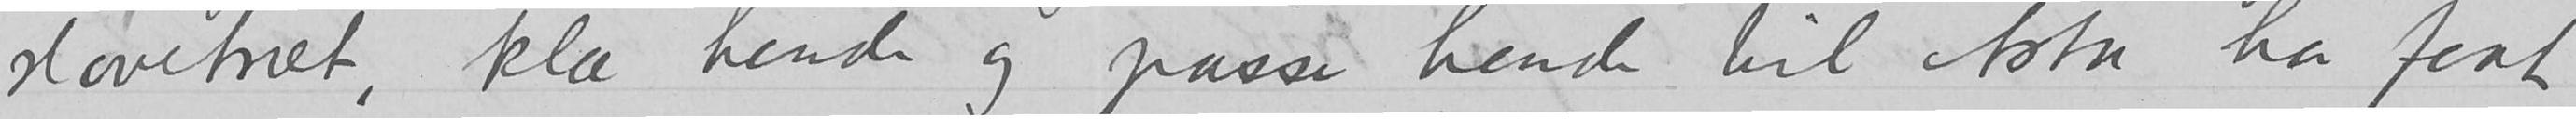sløvetræt, klæ hende og passe hende til Asta har faat
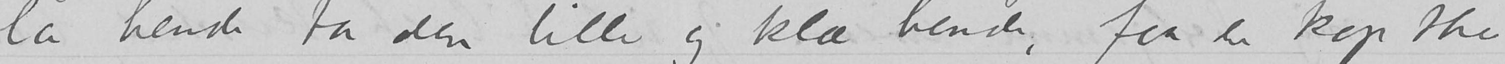la hende ta den lille og klæ hende, faa en kop the
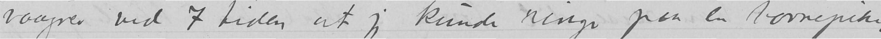vaagner ved 7 tiden at jeg kunde ringe paa en barnepike,
